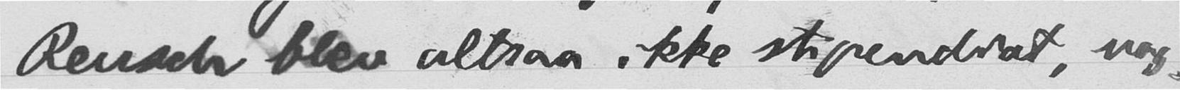Reusch blev altsaa ikke stipendiat, uag-
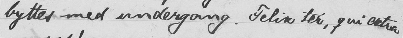byttes med undergang. Felix ter, qui extra
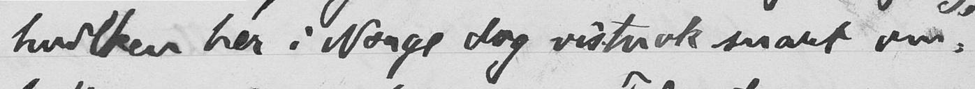hvilken her i Norge dog vistnok snart om-
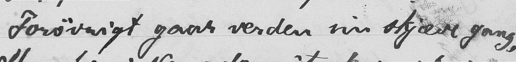Forøvrigt gaar verden sin skjæve gang,
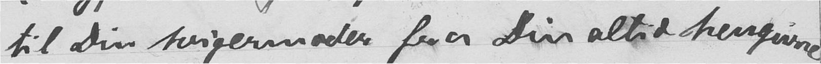til Din svigermoder fra Din altid hengivne
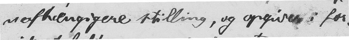uafhængigere stilling, og opgiver i for-
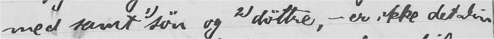med samt 1) søn og 2) døttre, - er ikke det Din
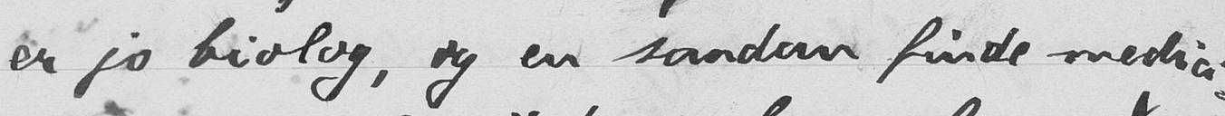er jo biolog, og en saadan finde medici-
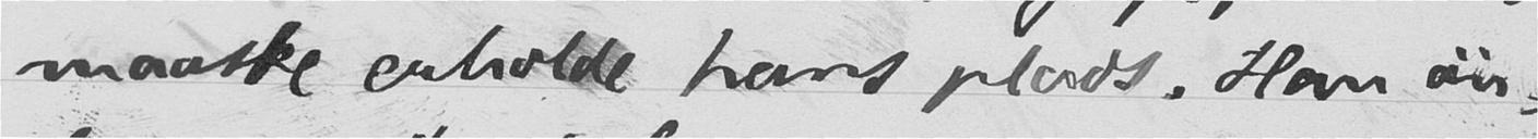maaske erholde hans plads. Han øn-
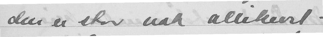den er stor nok allikevel.
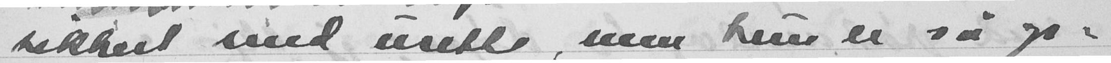sikkert med urette, men hun er så op-
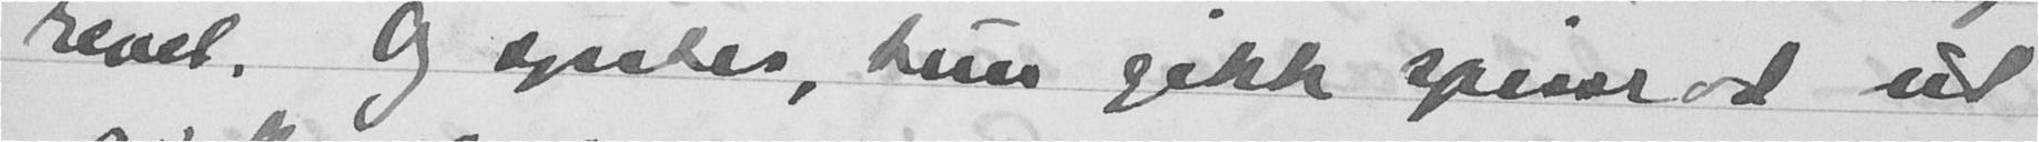revet. Og syntes, hun gikk spissrod ud
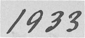1933
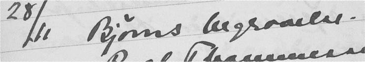28/11 Bjørns begravelse.
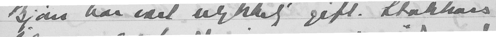Bjørn har vært ulykkelig gift. Stakkars
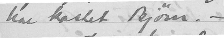har kostet Bjørn. -
